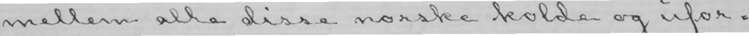mellem alle disse norske kolde og ufor-
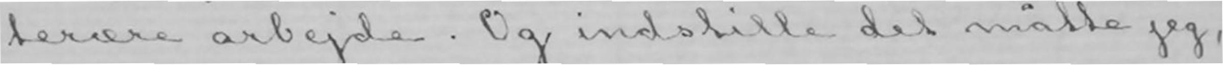terære arbejde. Og indstille det måtte jeg,
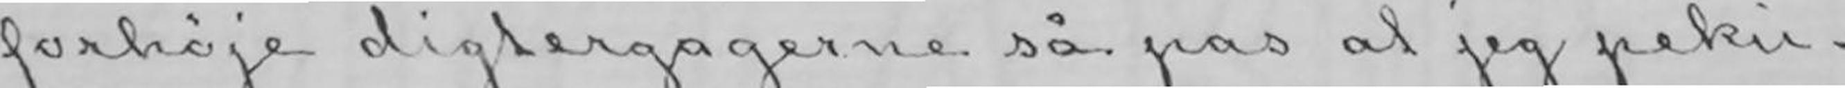forhøje digtergagerne så pas at jeg peku-
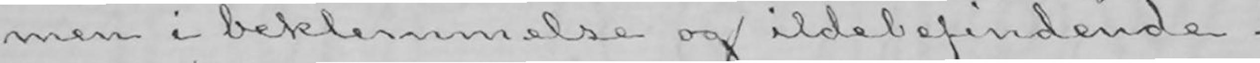men i beklemmelse og ildebefindende.
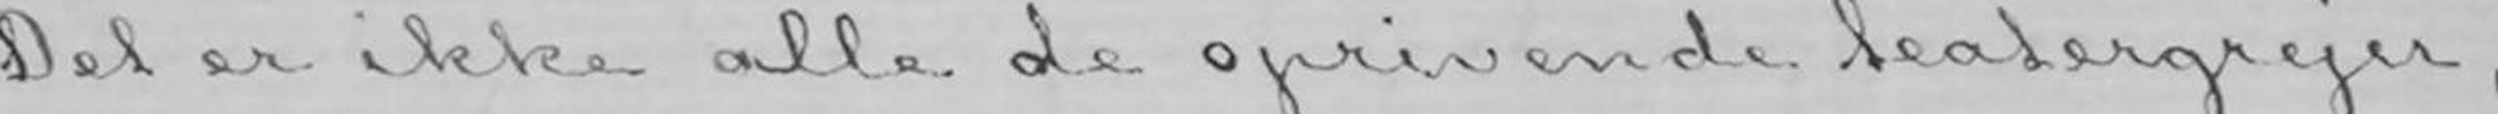Det er ikke alle de oprivende teatergrejer,
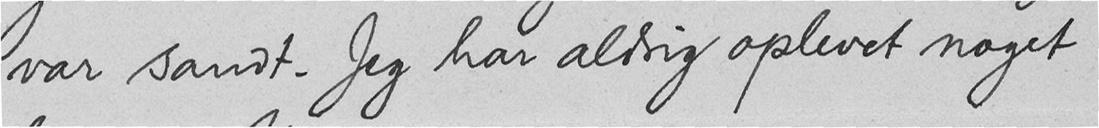var sandt. Jeg har aldrig oplevet noget
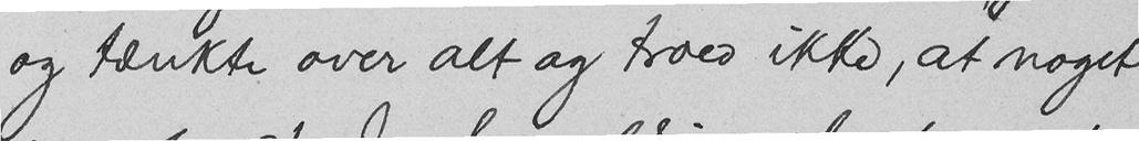og tænkte over alt og troed ikke, at noget
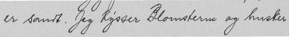er sandt. Jeg kysser Blomsterne og husker
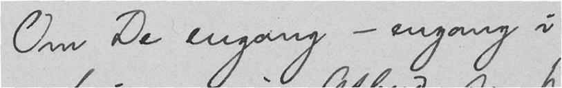Om De engang - engang i
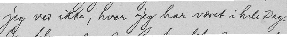jeg ved ikke, hvor jeg har været i hele Dag.
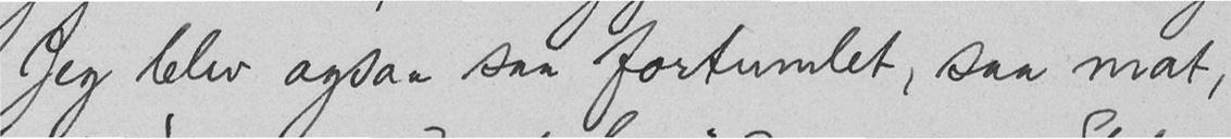Jeg blev ogsaa saa fortumlet, saa mat,
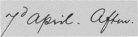7d April. Aften.
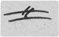#
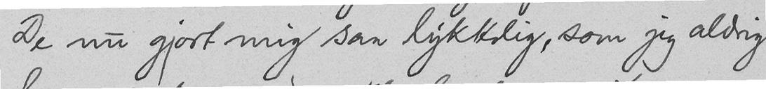De nu gjort mig saa lykkelig, som jeg aldrig
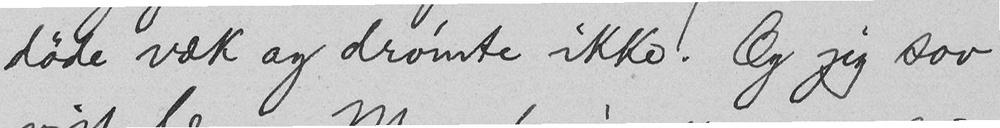døde væk og drømte ikke. Og jeg sov
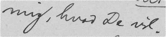mig, hvad De vil.
